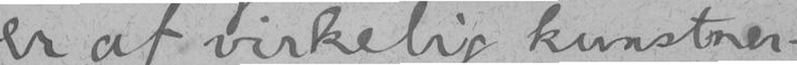er af virkelig kunstner-
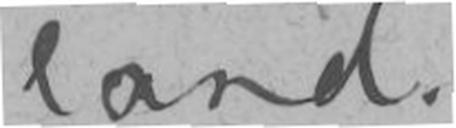land.
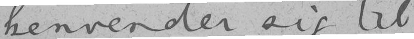henvender sig til
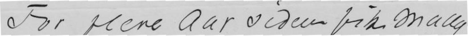For flere Aar siden fik Mally
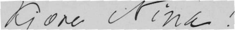Kjære Nina!
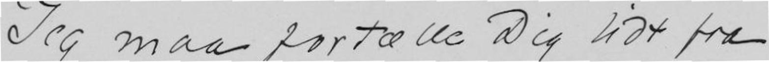Jeg maa fortælle Dig lidt fra
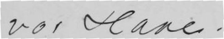vor have.
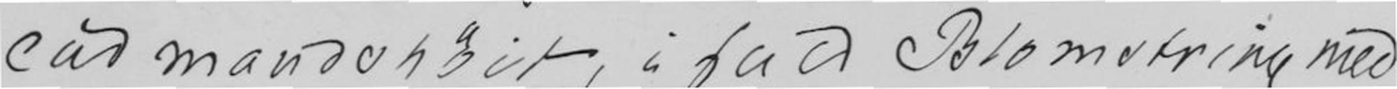mandshøit, i fa Blomstring med
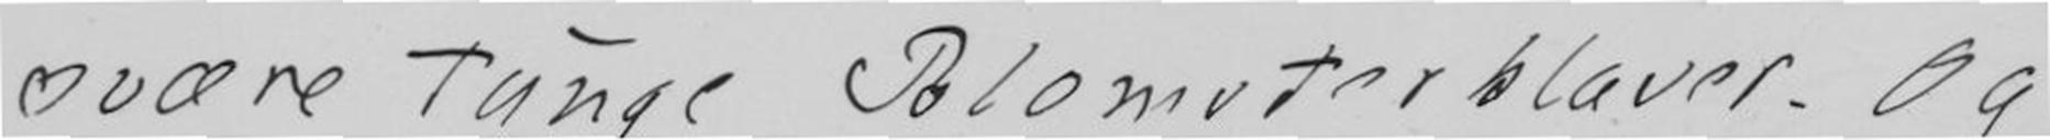svære Tunge Blomsterklaver. og
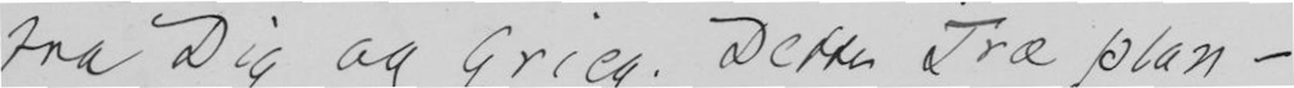fra Dig og Grieg. Dette Træ plan-
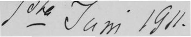1ste Juni 1911
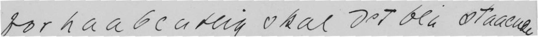forhaabentlig skal det bli staaende
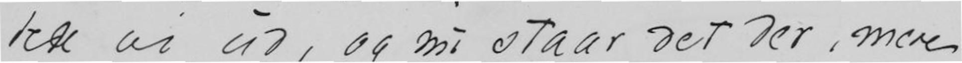tede vi ud, og nu staar det der, mere
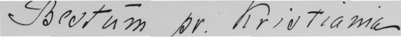Bestum pr. Kristiania
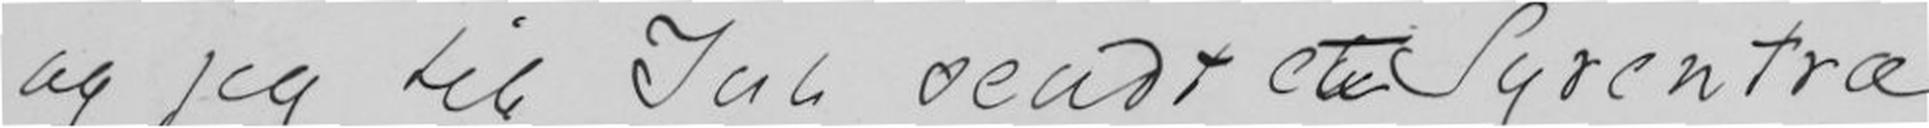og jeg til sendt et Syrentræ
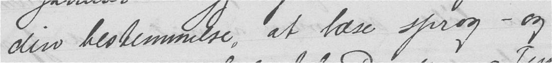din bestemmelse, at læse sprog - og
